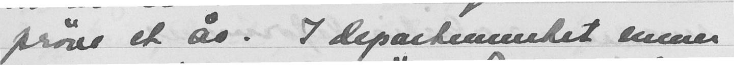prøve et år. I departementet mener
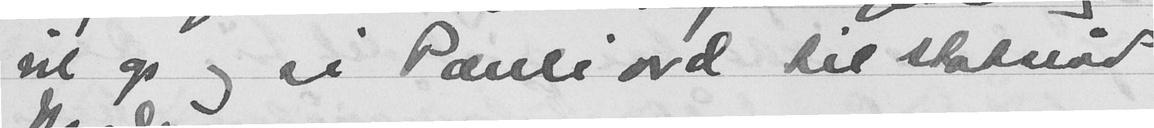vil op og si Pauli ord til statsråd
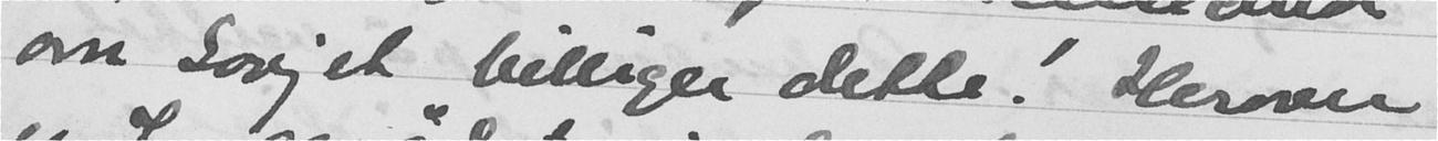om Sovjet billiger dette! Herover
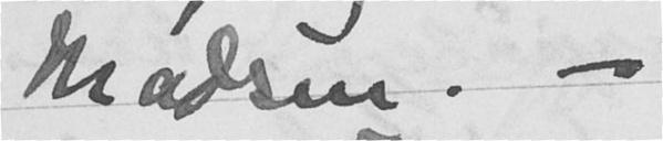Madsen. -
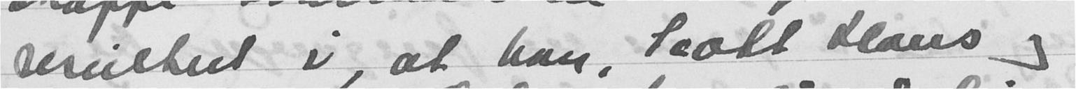resultert i, at han, Scott Hans og
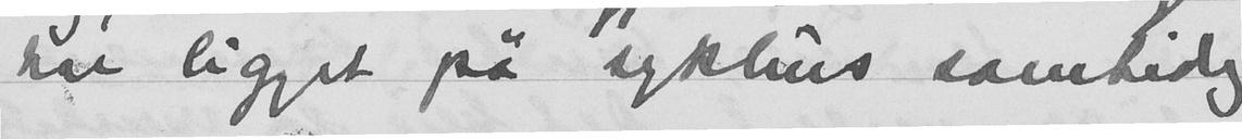har ligget på sykehus samtidig
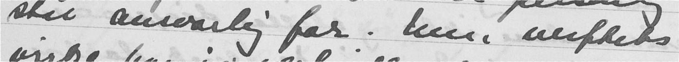står ansvarlig for. Men verftets
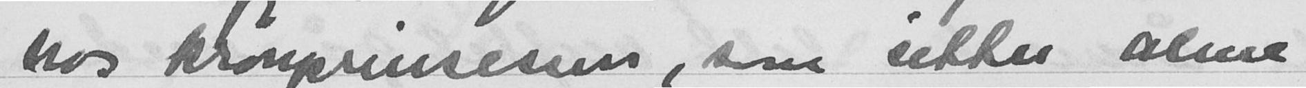hos kronprinsessen, som sitter alene
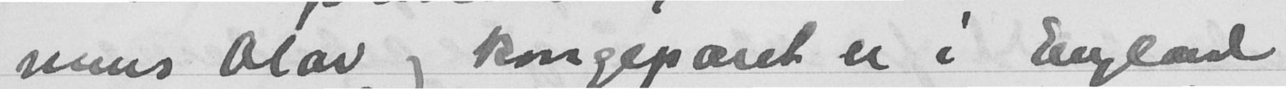mens Olav og kongeparet er i England
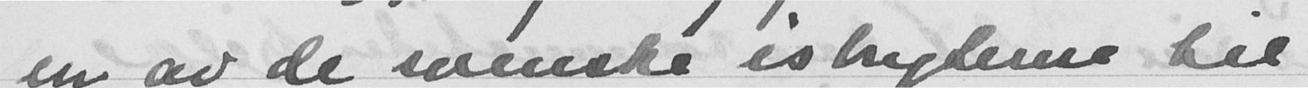en av de svenske isbryterne til
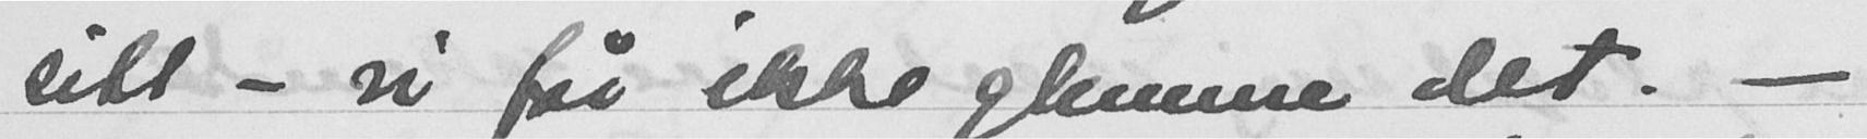sitt - vi får ikke glemme det. -
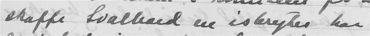skaffe Svalbard en isbryter har
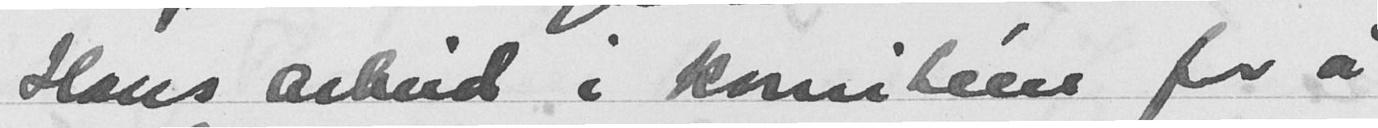Hans arbeid i komitéen for å
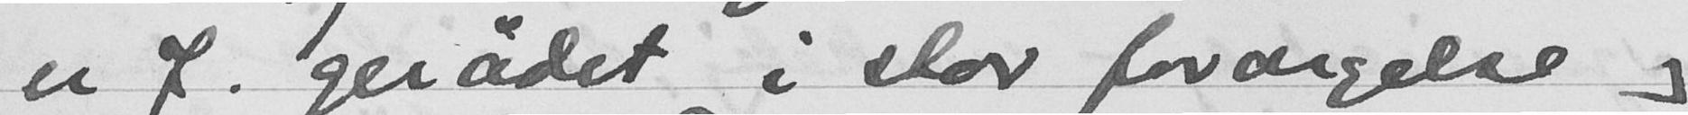er J. gerådet i stor forargelse og
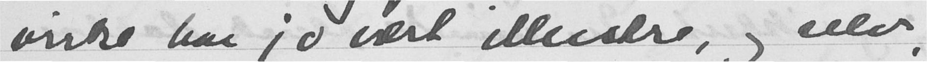virke har jo vært ellerstre, og selv
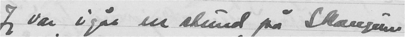Jeg var igår en stund på Skaugum
